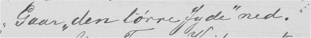"Gaar "den tørre jyde" ned."
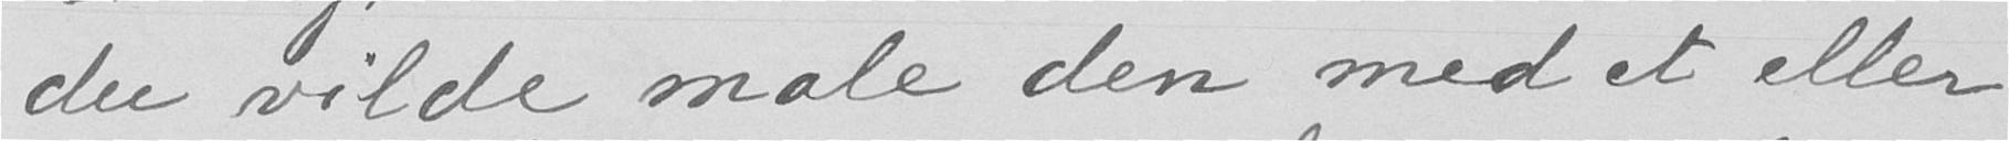du vilde male den med et eller
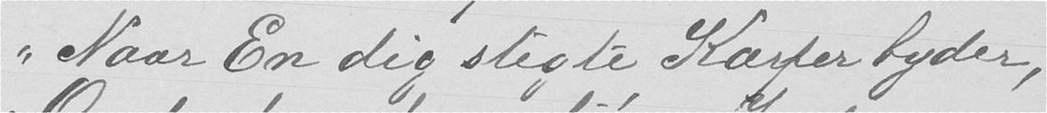"Naar En dig stegte Karper byder,
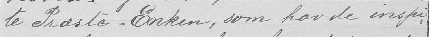te Præste-Enken, som havde inspi-
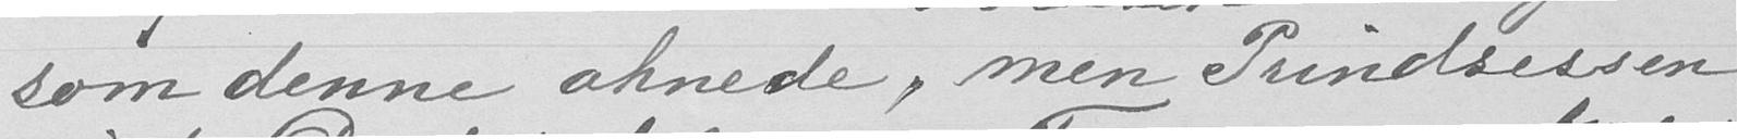som denne ahnede, men Prindsessen
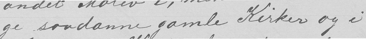ge saadanne gamle Kirker og i
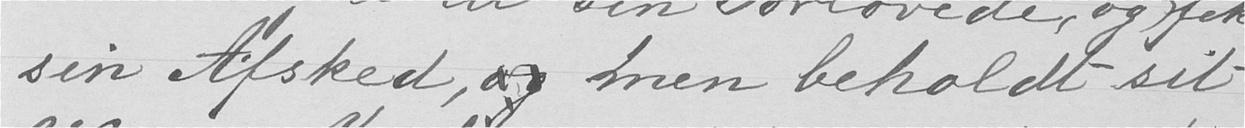sin Afsked, og fruen beholdt sit
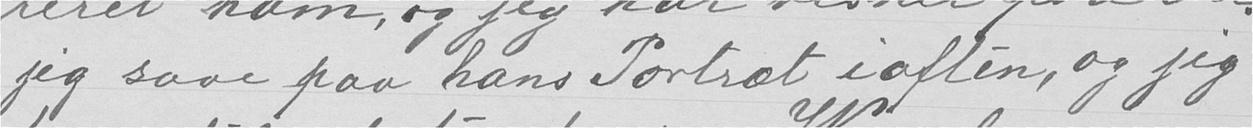jeg saae paa hans Portræt iaften, og jeg
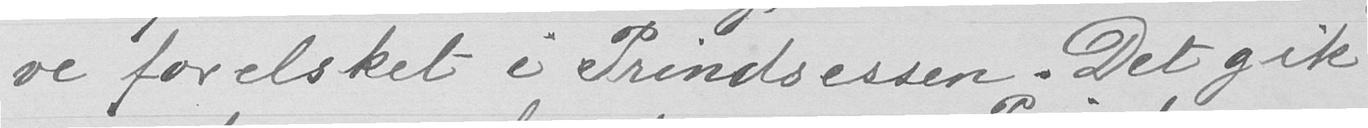ve forelsket i Prindsessen. Det gik
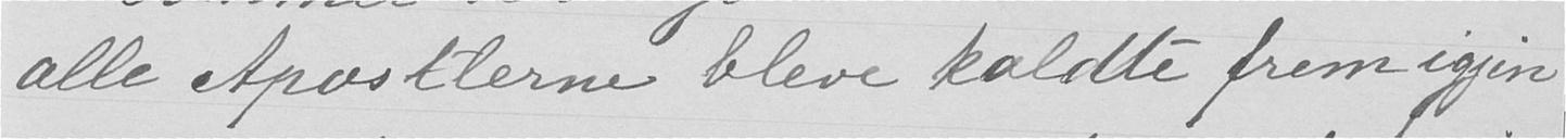alle Apostlerne bleve kaldte frem igjen
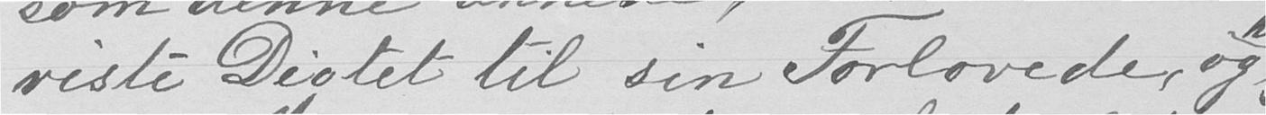viste Digtet til sin Forlovede, og
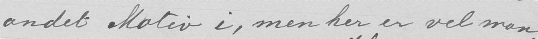andet Motiv i, men her er vel man-
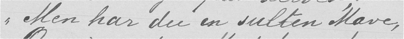"Men har du en sulten Mave,
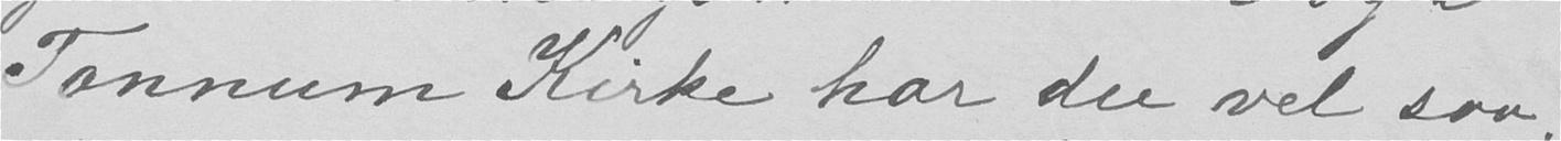Tannum Kirke har du vel saa-
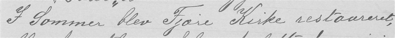I Sommer blev Fjære Kirke restaureret,
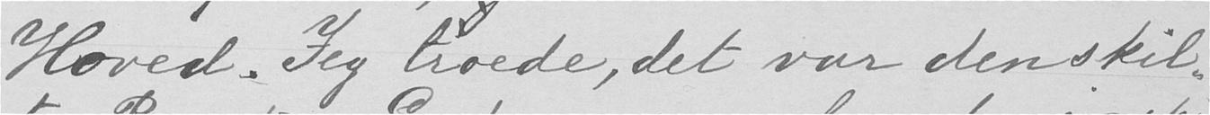Hoved. Jeg troede, det var den skil-
